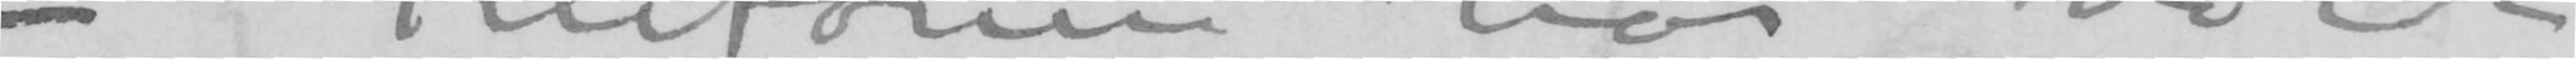– Telefonen har været
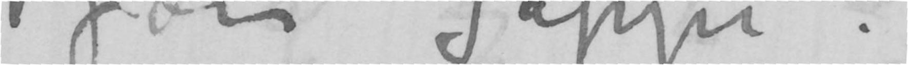Kjære Jappe!
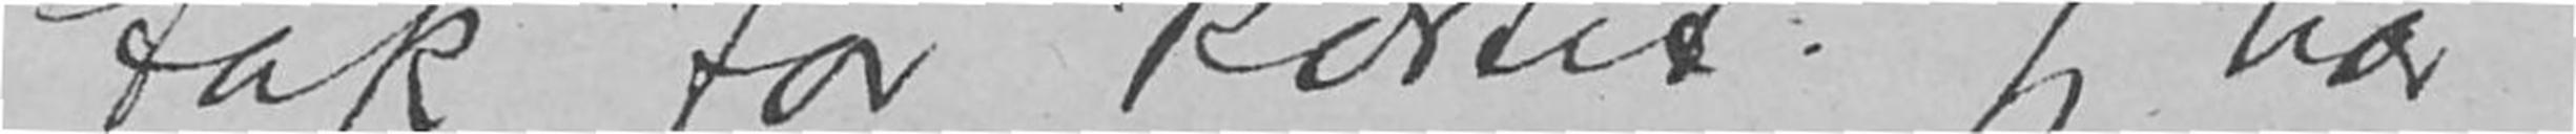tak for kortet. Jeg har
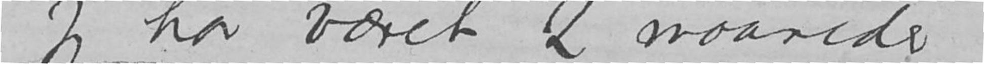Jeg har været 2 maaneder
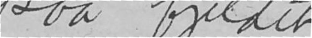paa fjeldet.
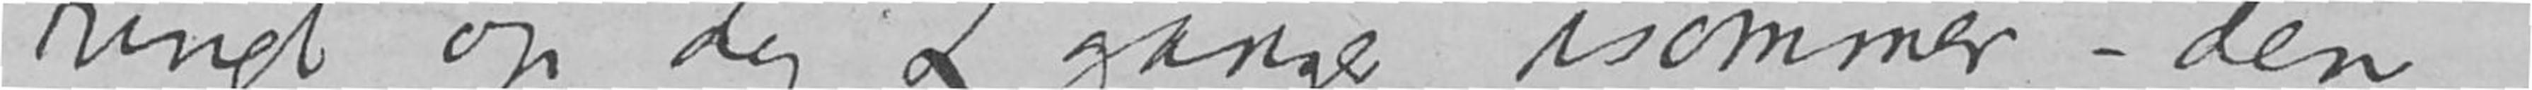ringt op dig 2 ganger isommer – den
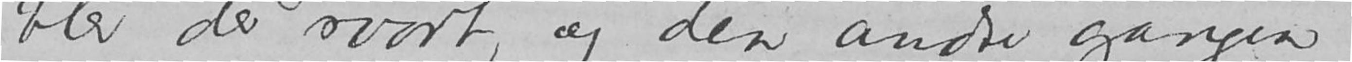blev der svart, og den andre gangen
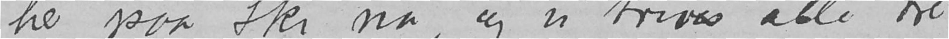her paa Ski nu, og vi trives alle tre –
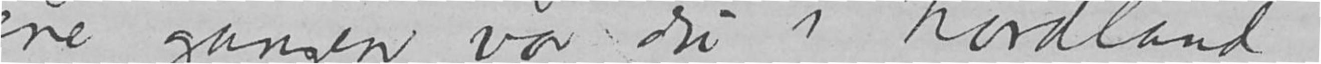ene gangen var du i Nordland,
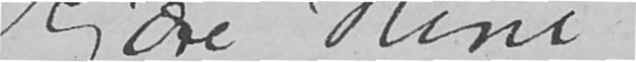Kjære Nini,
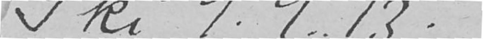Ski 9.9.13.
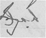toget
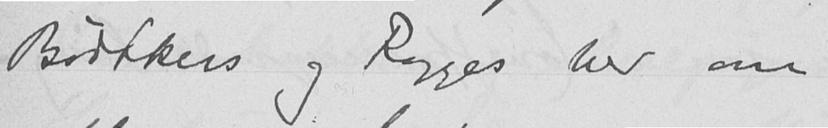Bødtkers og Rogges her om
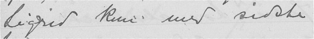Sigrid kom med sidste
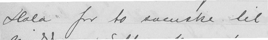Hola for to svenske til
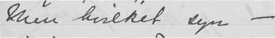Men hvilket syn -
